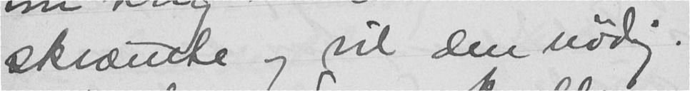skræmte og vil den nødig.
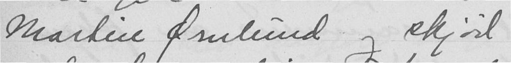Martin Ørnlund og skjød
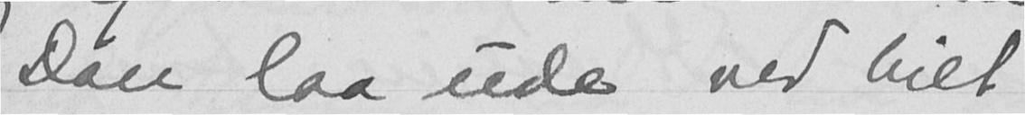Don laa ude ved hiet
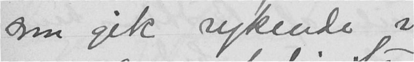som gik rykende i
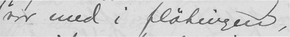var med i fløtingen,
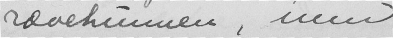rævehunnen, men
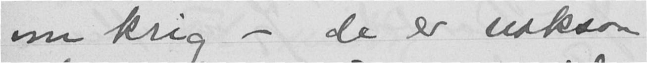om krig - de er noksaa
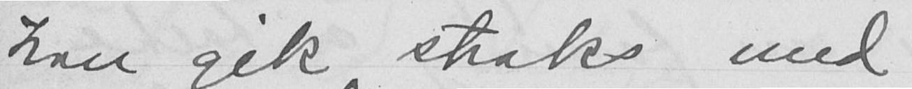han gik straks med
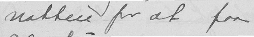natten for at faa
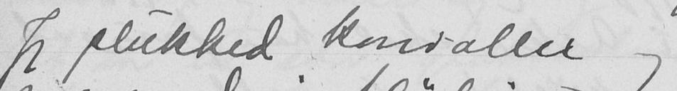Jeg plukked konvaller og
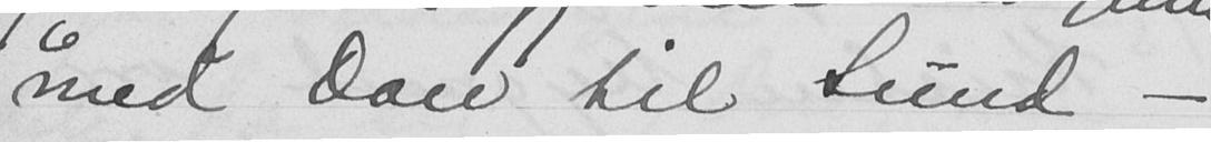med Don til Sund -
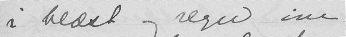i blæst og regn om
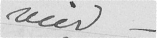veir -
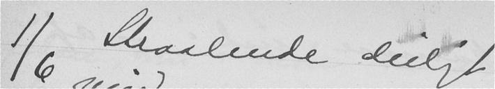1/6 Straalende deiligt
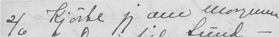2/6 Kjørte jeg om morgenen
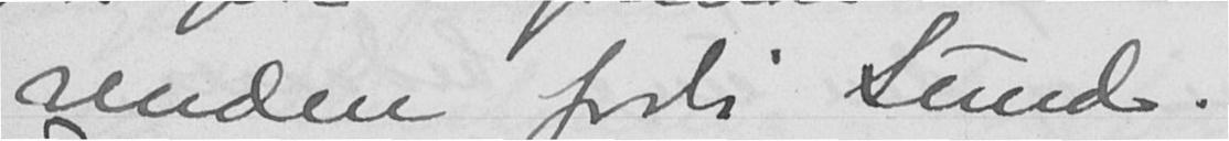renden forbi Sund.
